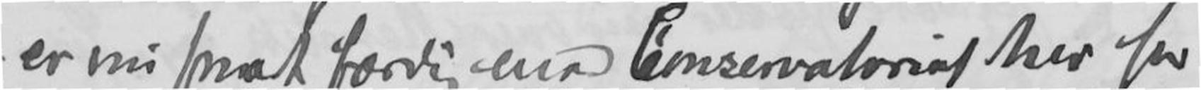er nu snart færdig med Conservatoriet her for
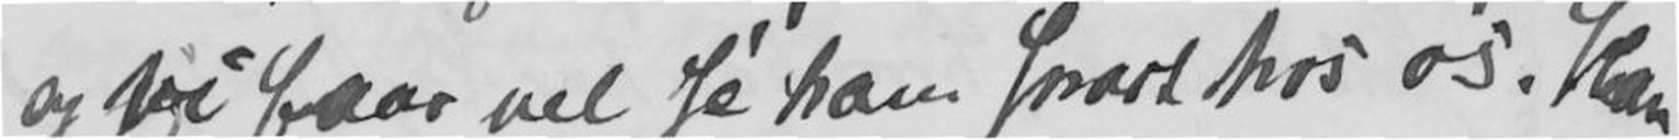og jeg faar vel se ham snart hos os. Han
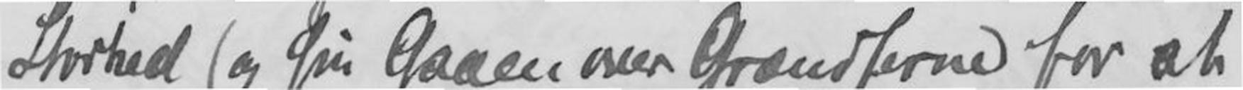Storhed og sin Gaaen over Grændserne for at
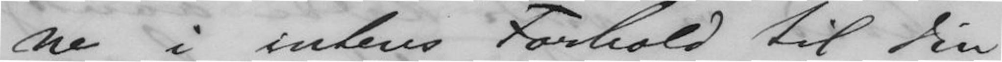ne i intens Forhold til Din
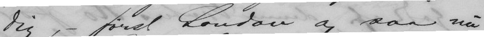Dig, - Først London og saa nu
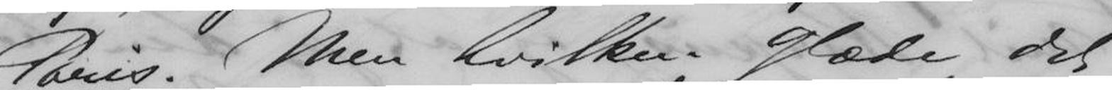Paris. Men hvilken glæde det
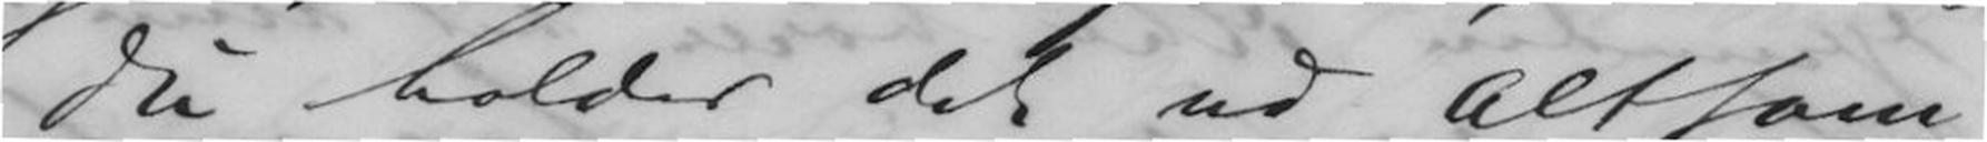Du holder det ud Altsam-
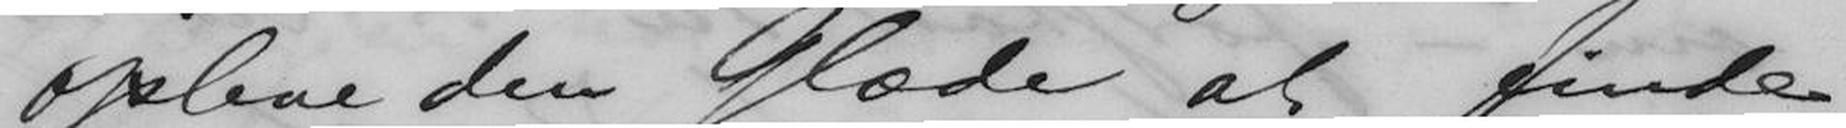opleve den Glæde at finde
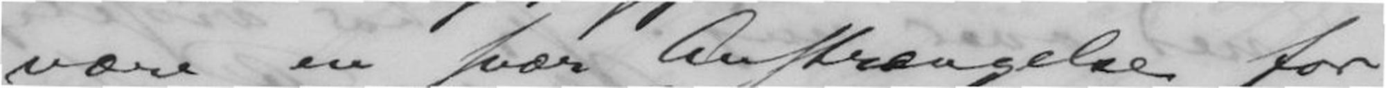være en svær Anstrængelse for
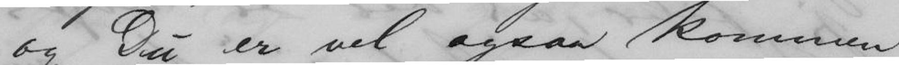og Du er vel ogsaa kommen
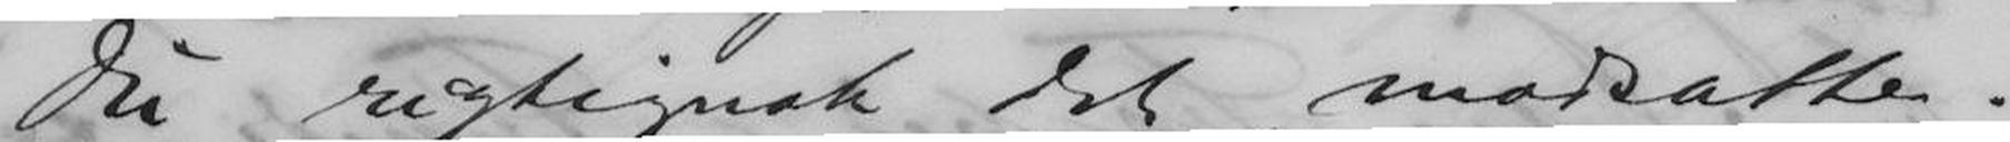Du rigtignok det modsatte.
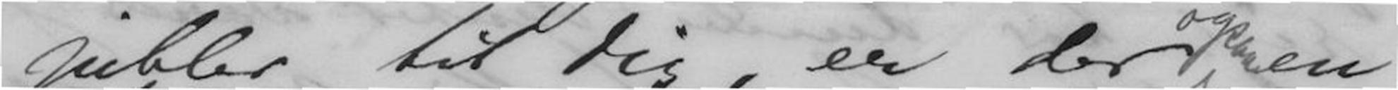jubler til dig, er der ogsaa en-
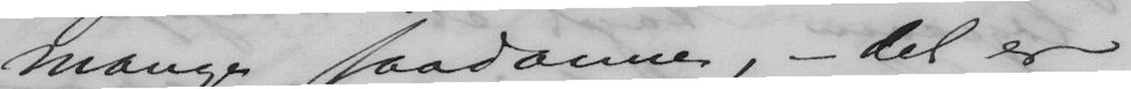mange saadanne, - det er
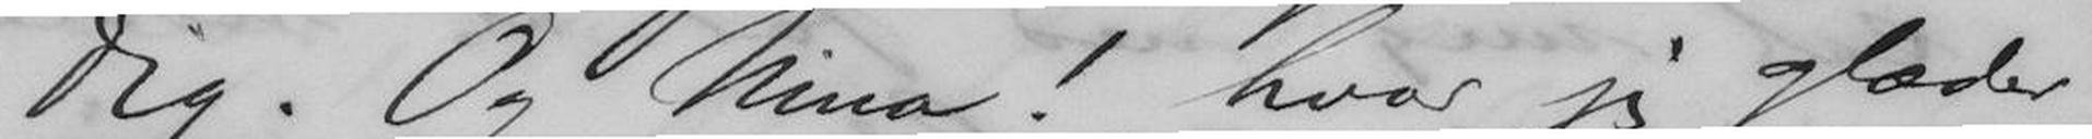Dig. Og Nina! hvor jeg glæder
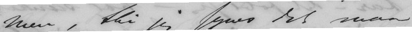men, thi jeg synes det maa
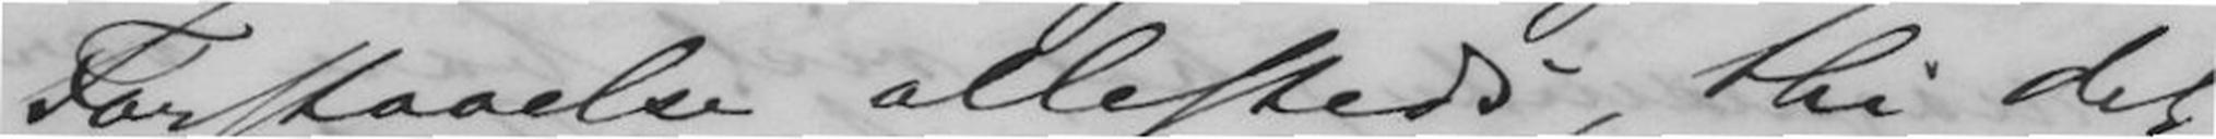Forstaalse allesteds, thi det
